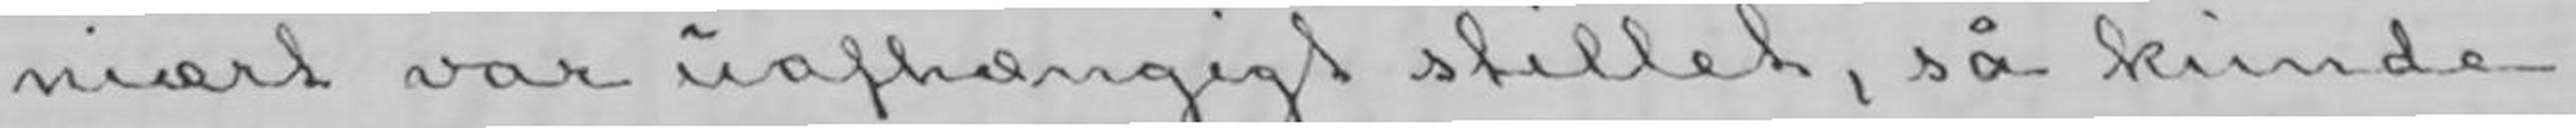niært var uafhængigt stillet, så kunde
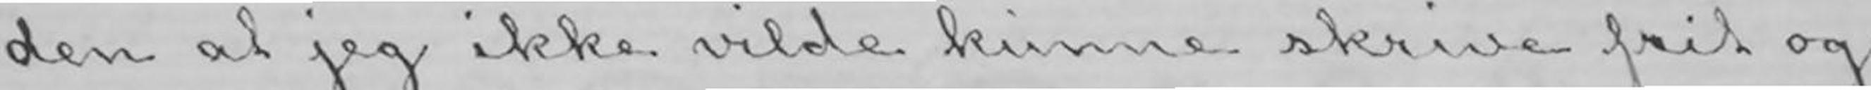den at jeg ikke vilde kunne skrive frit og
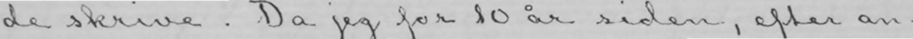de skrive. Da jeg for 10 år siden, efter an-
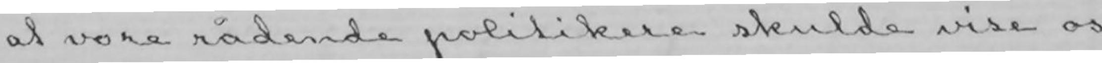at vore rådende politikere skulde vise os
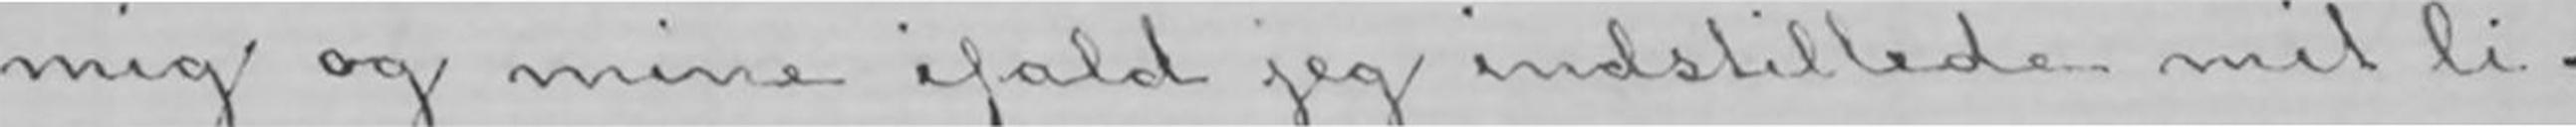mig og mine ifald jeg indstillede mit li-
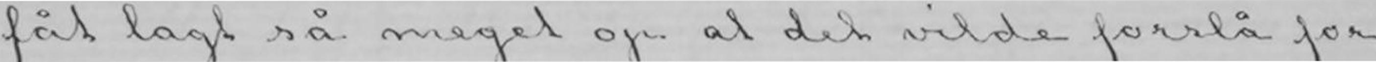fåt lagt så meget op at det vilde forslå for
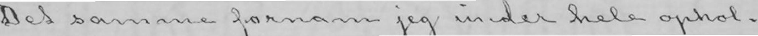Det samme fornam jeg under hele ophol-
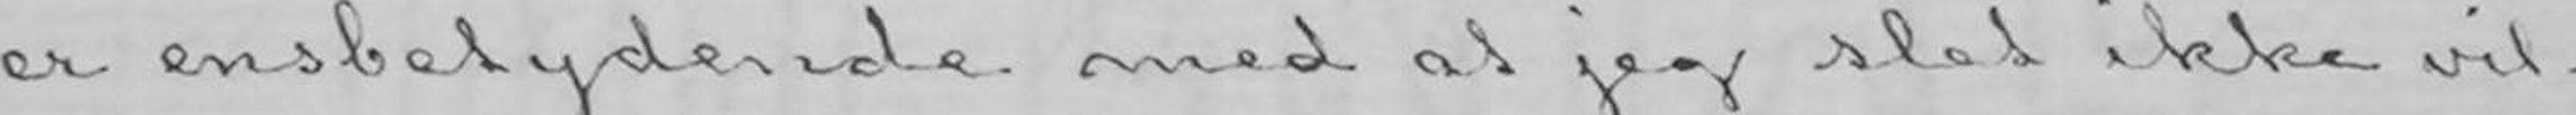er ensbetydende med at jeg slet ikke vil-
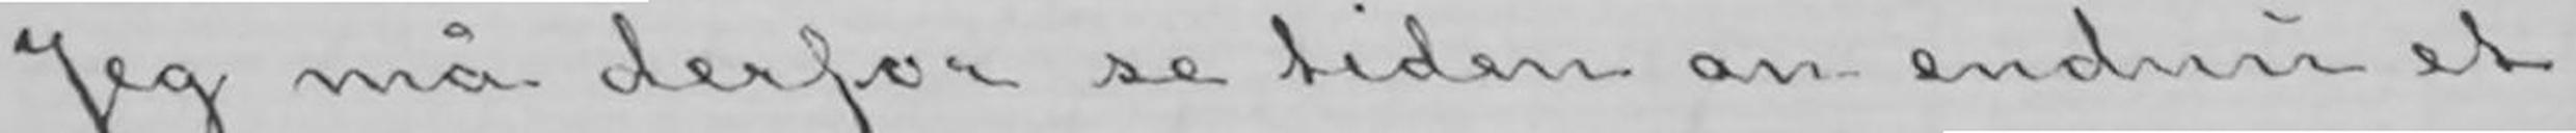Jeg må derfor se tiden an endnu et
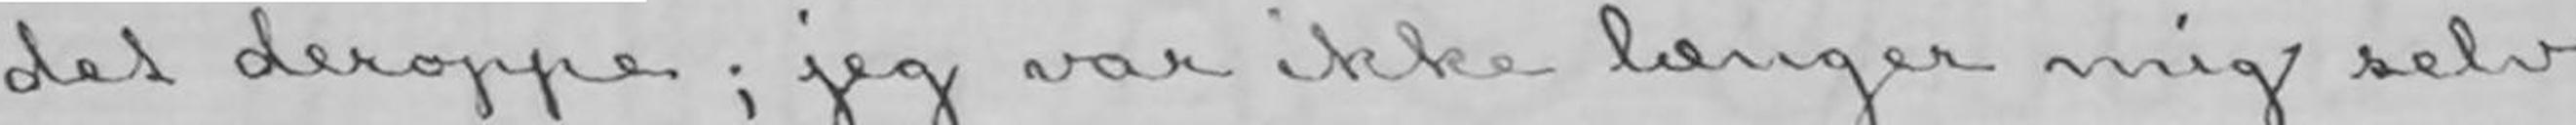det deroppe; jeg var ikke længer mig selv
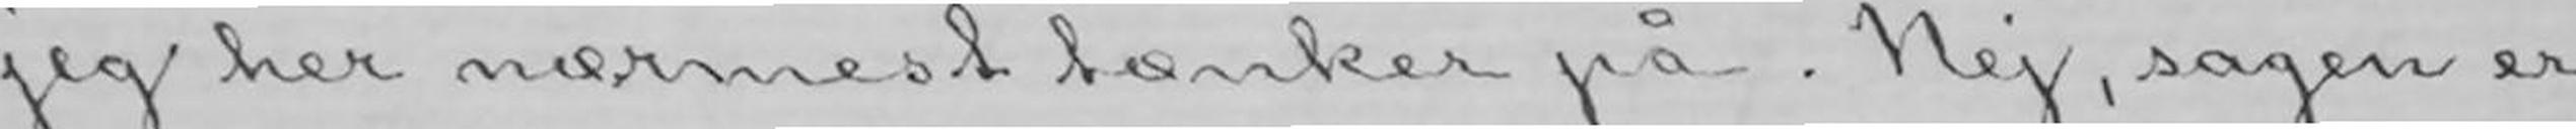jeg her nærmest tænker på. Nej, sagen er
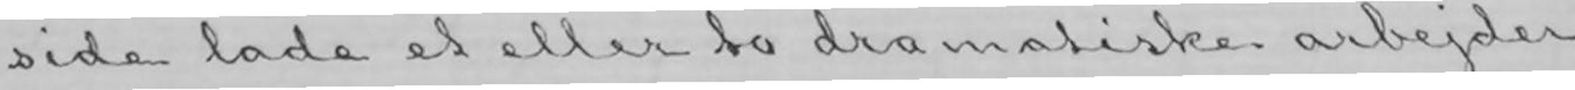side lade et eller to dramatiske arbejder
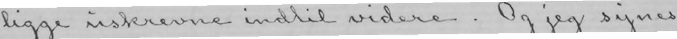ligge uskrevne indtil videre. Og jeg synes
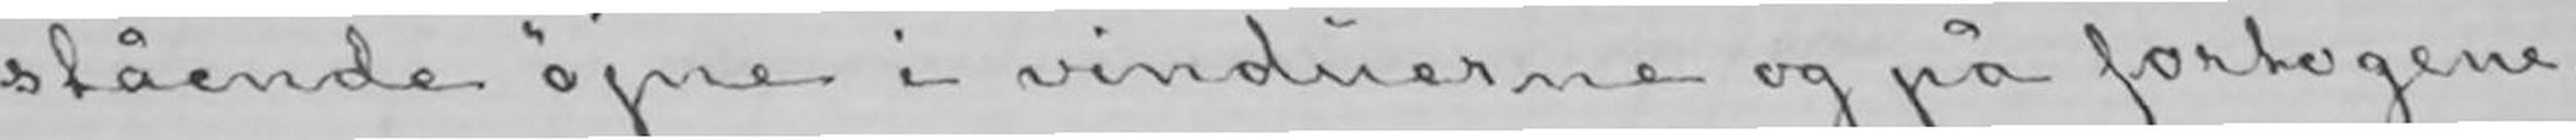stående øjne i vinduerne og på fortogene.
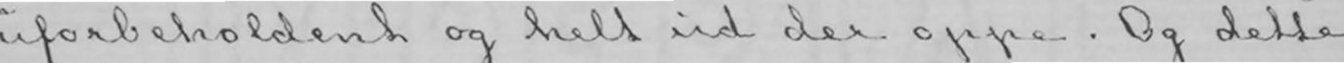uforbeholdent og helt ud der oppe. Og dette
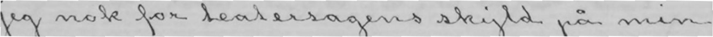jeg nok for teatersagens skyld på min
